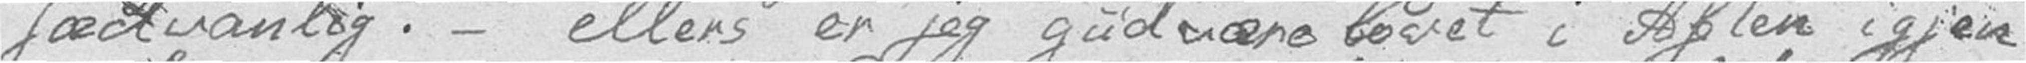sædvanlig. – ellers er jeg gud være lovet i Aften igjen
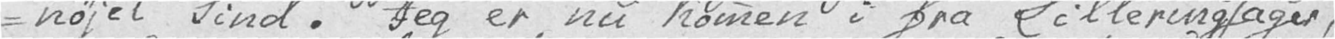-nøjet Sind. Jeg er nu kommen i fra Lilleringsager,
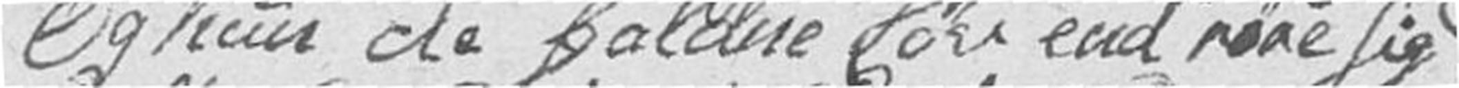Og kun de faldne Løv end røre sig,
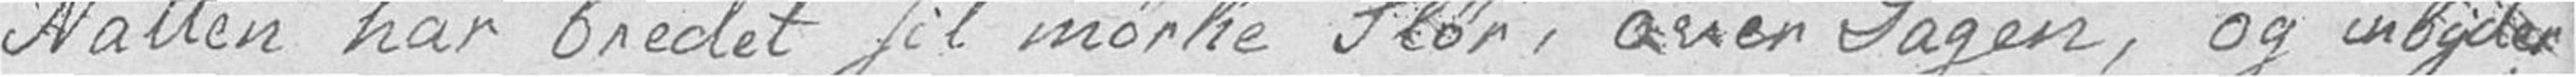Natten har bredet sit mørke Slør, over Dagen, og inbyder
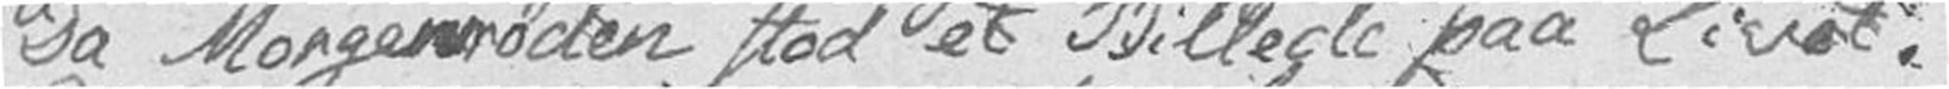Da Morgenrøden stod et Billede paa Livet.
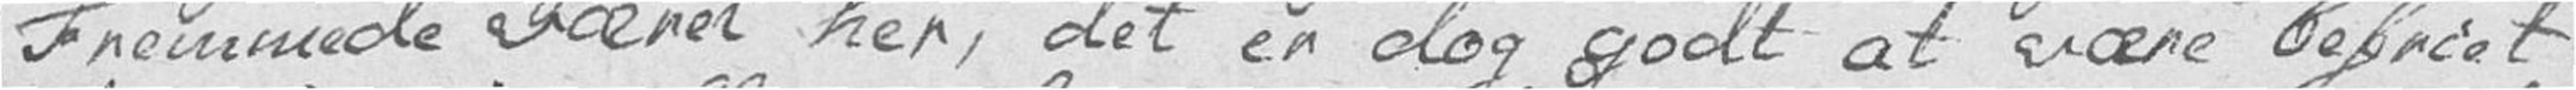Fremmede været her, det er dog godt at være befriet
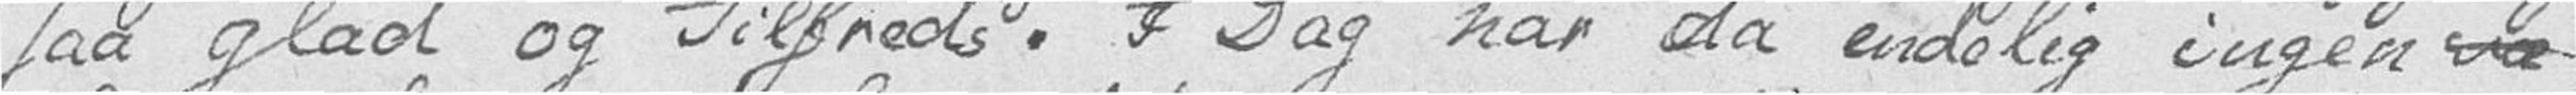saa glad og Tilfreds. I Dag har da endelig ingen væ
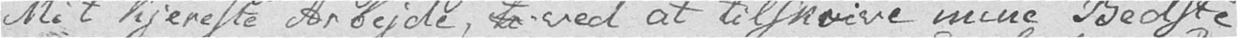Mit kjereste Arbejde, ti ved at tilskrive mine Bedste
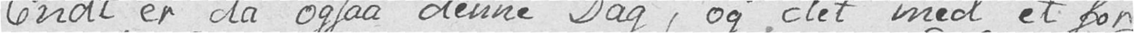Endt er da ogsaa denne Dag, og det med et for
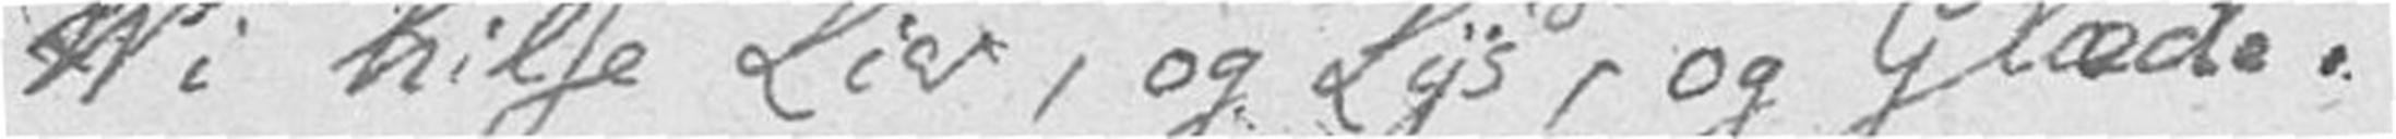Vi hilse Liv, og Lys, og Glæde.
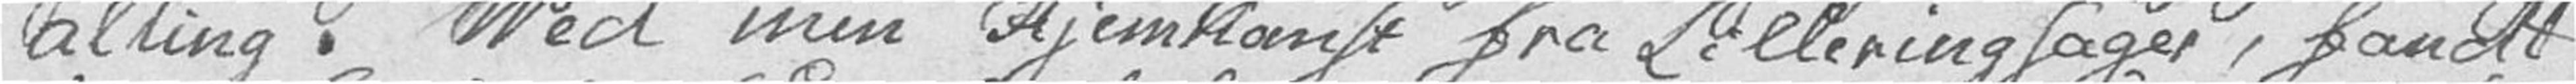alting. Ved min Hjemkomst fra Lilleringsager, fandt
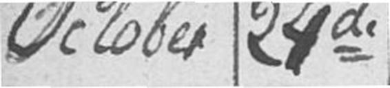October 24de
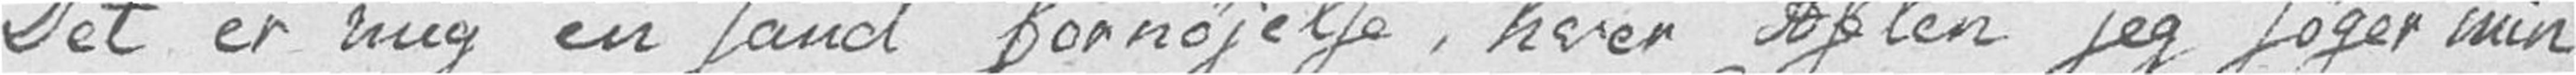Det er mig en sand fornøjelse, hver Aften jeg søger min
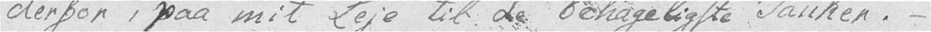derfor, paa mit Leje til de behageligste Tanker. –
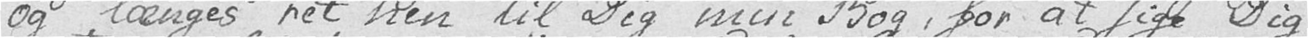og længes ret hen til Dig min Bog, for at sige Dig
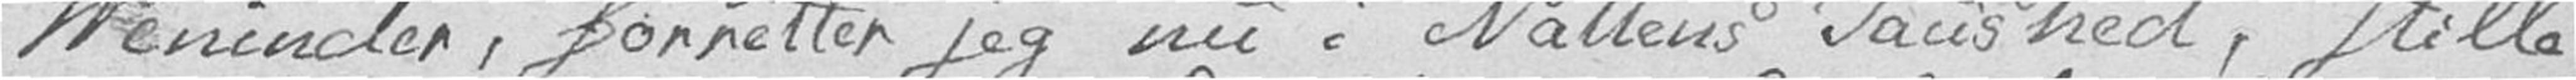Veninder, forretter jeg nu i Nattens Taushed, stille
